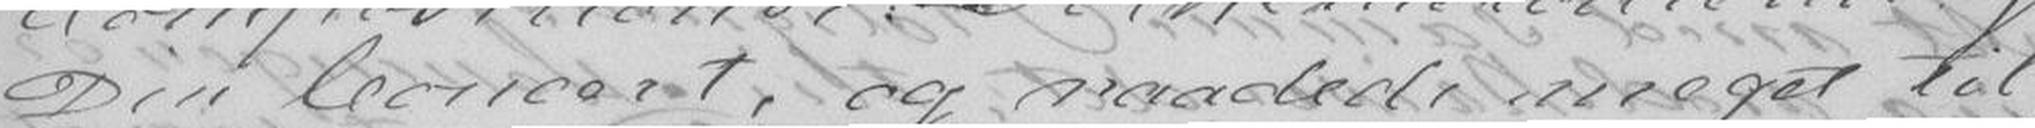Din Concert, og raadede meget til
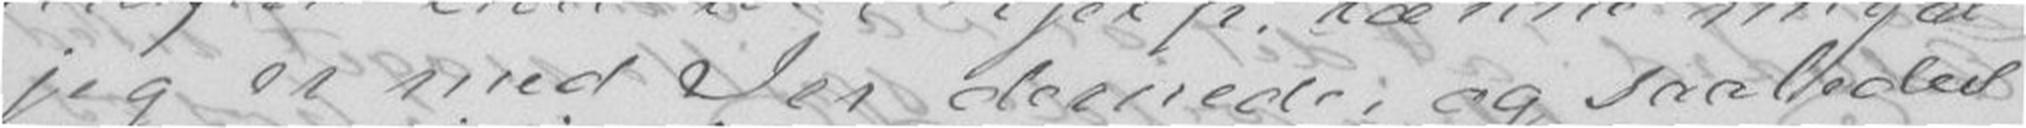jeg er med Jer dernede; og saaledes
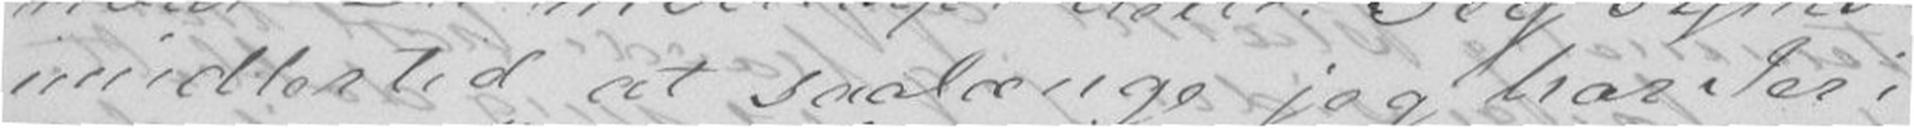imidlertid at saalænge jeg har Jer i
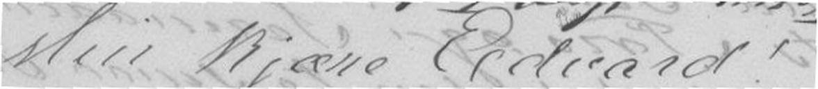Min kjære Edvard!
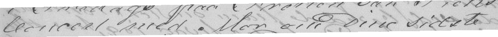Concert med Mor om Din sidste
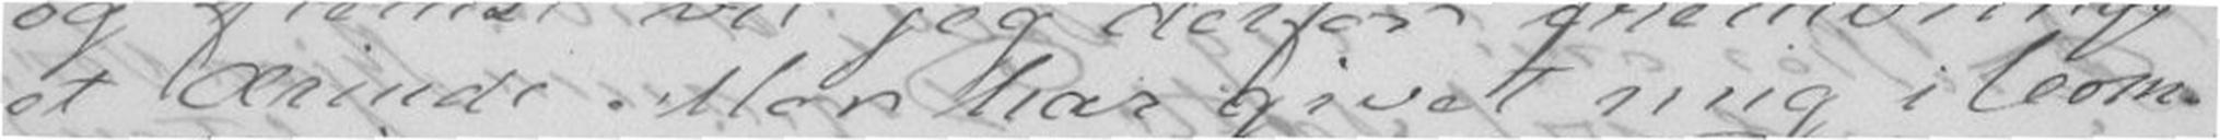et Ærinde Mor har givet mig i Com-
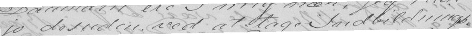jo desuden ved at tage Indbildnings-
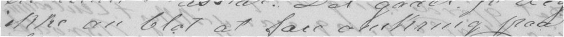ikke an blot at fare omkring paa
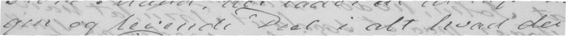gen og levende Deel i alt hvad der
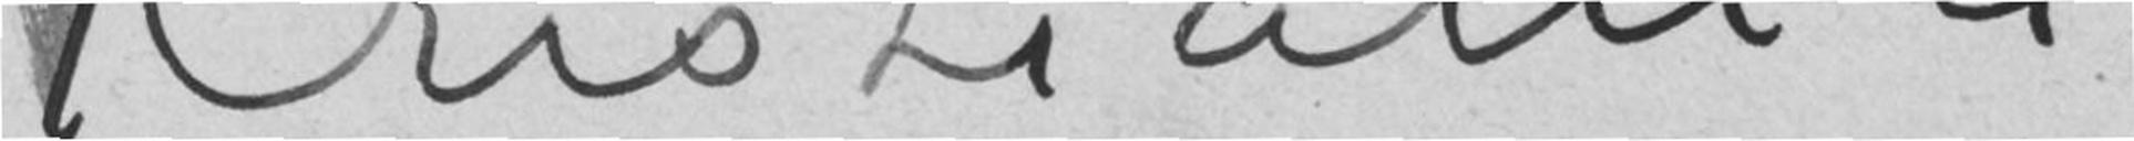Kristiania
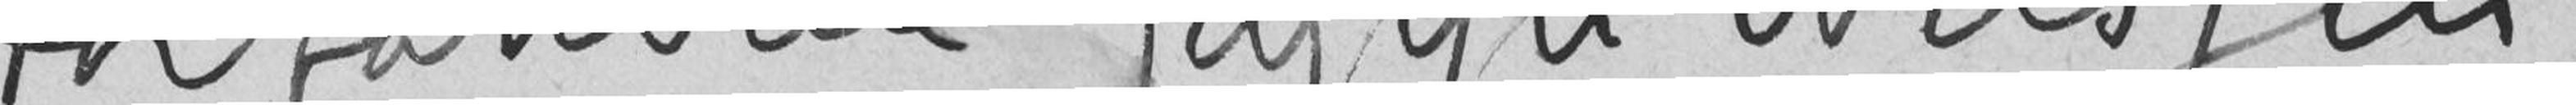Forfatteren Jappe Nilssen
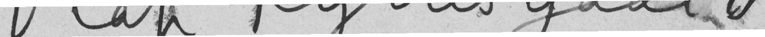Olaf Kyrres gade 11
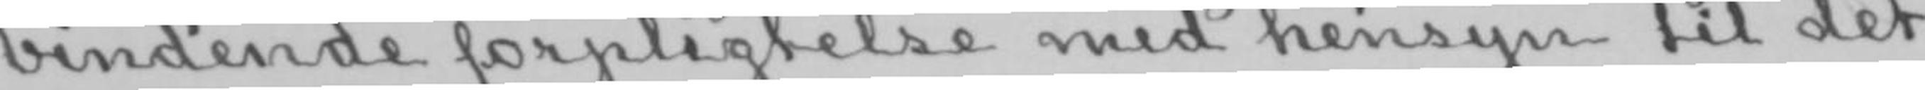bindende forpligtelse med hensyn til det
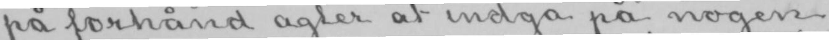på forhånd agter at indgå på nogen
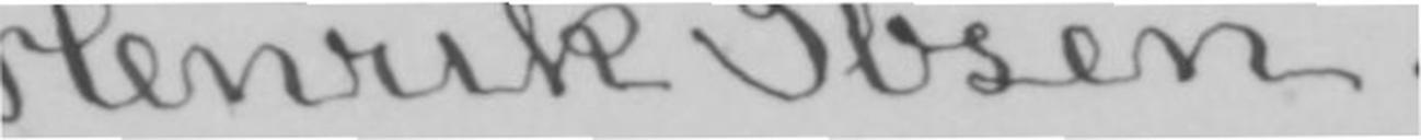Henrik Ibsen.
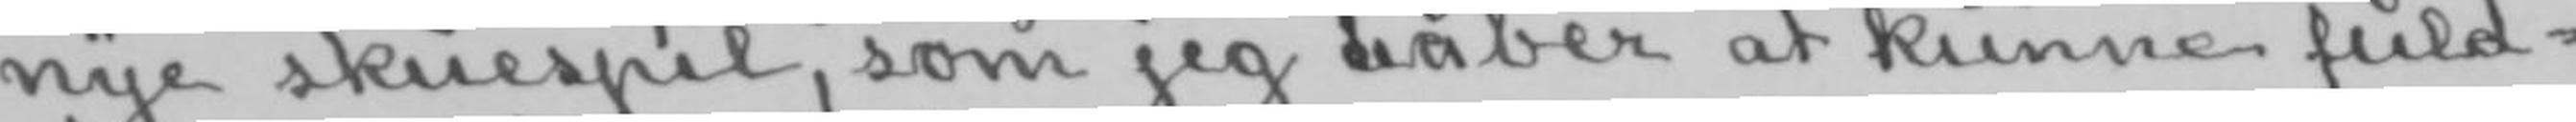nye skuespil, som jeg håber at kunne fuld-
